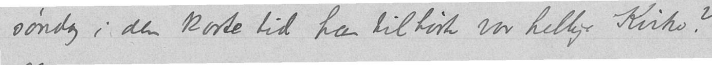søndag i den korte tid han tilhørte vor hellige Kirke?
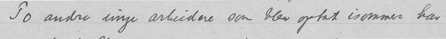To andre unge arbeidere som blev optat isommer har
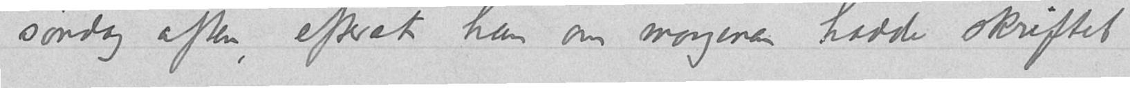søndag aften, efterat han om morgenen hadde skriftet
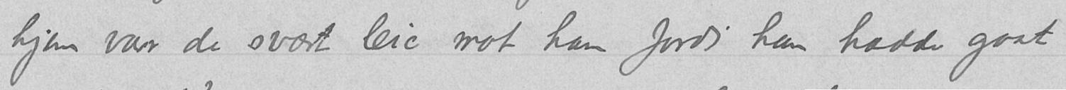hjem var de svært leie mot ham fordi han hadde gaat
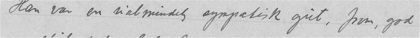Han var en ualmindelig sympatisk gut, from, god
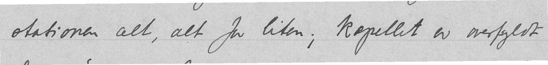stationen alt, alt for liten; kapellet av overfyldt
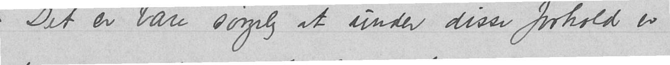– Det er bare sørgelig at under disse forhold er
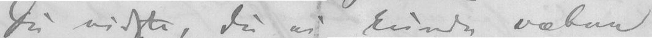Du vidste, du ei kunde væbne
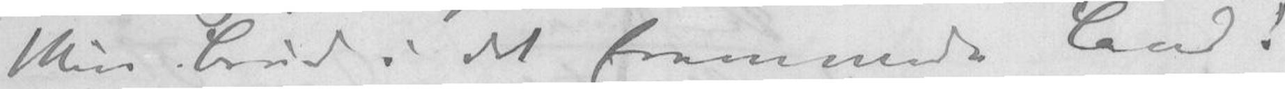Min Brud i det fremmede Land!
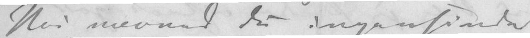Hvi nævned du ingensinde
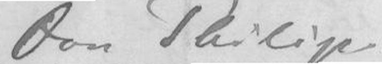Don Philip
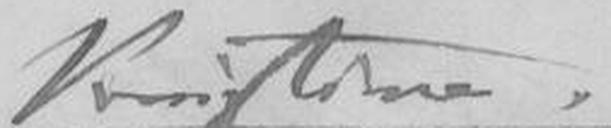Kristina.
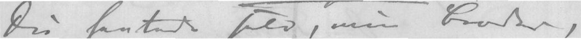Du hentede selv, min Broder,
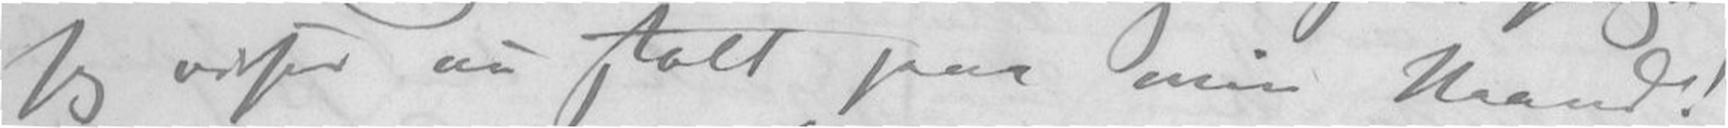Jeg viser nu stolt paa min Haand!
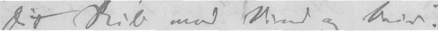Dit Skib mod Vind og Veir?
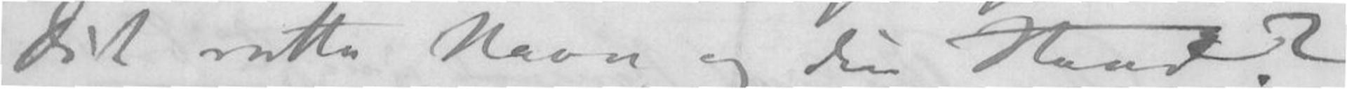Dit rette Navn og din Haand?
